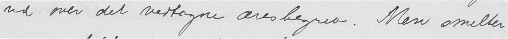ud over det vedtagne æresbegreb. Men smelter
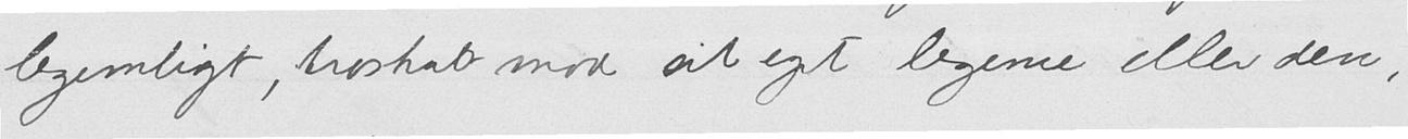legemligt, troskab mod sit eget legeme eller den,
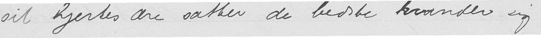sit hjertes ære sætter de bedste kvinder sig
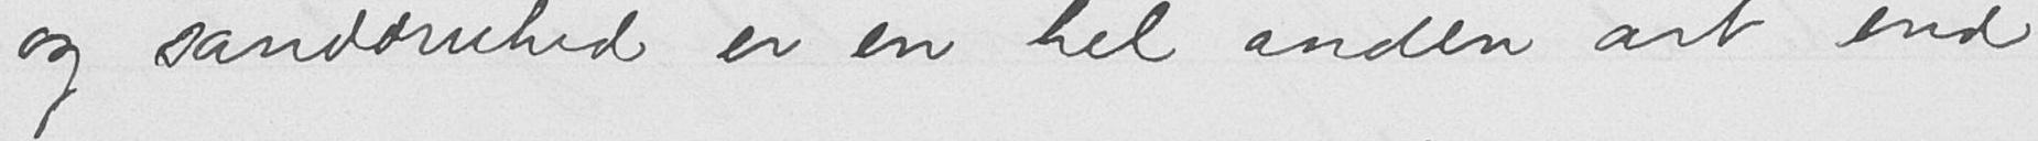og sanddruhed er en hel anden art end
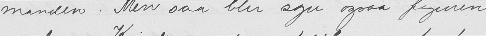manden. Men saa blir sgu ogsaa figuren
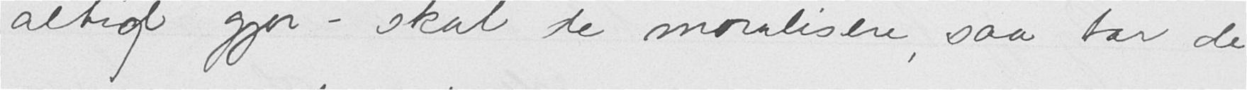altid gjør - skal de moralisere, saa tar de
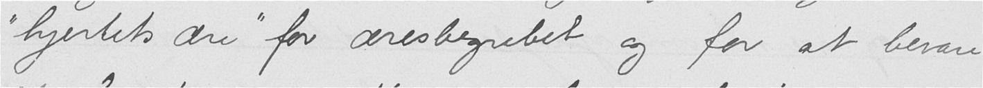"hjertets ære" for æresbegrebet og for at bevare
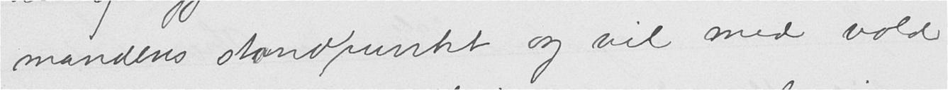mandens standpunkt og vil med vold
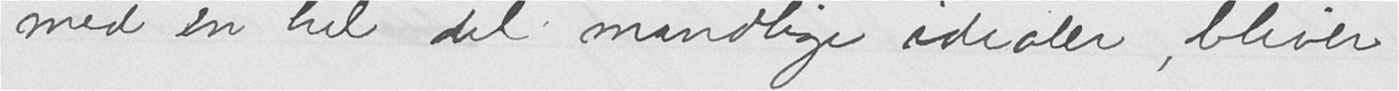med en hel del mandlige idealer, bliver
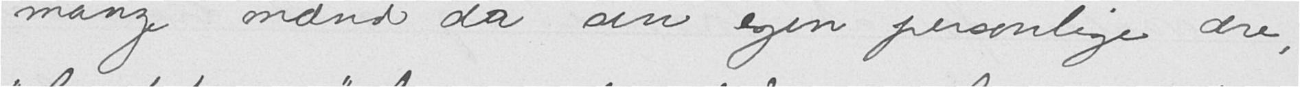mange mænd da sin egen personlige ære,
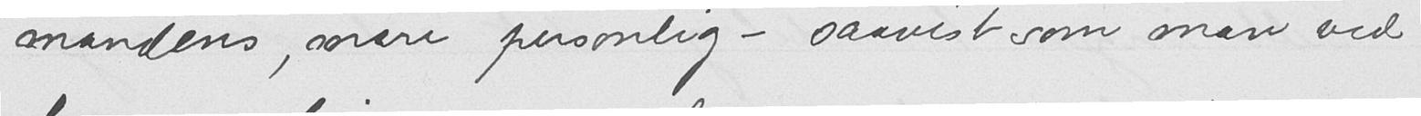mandens, mere personlig - saavist som man ved
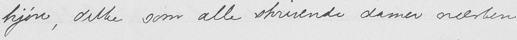kjøn, dette som alle skrivende damer næsten
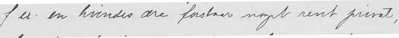f ex. en kvindes ære forstaar noget rent privat,
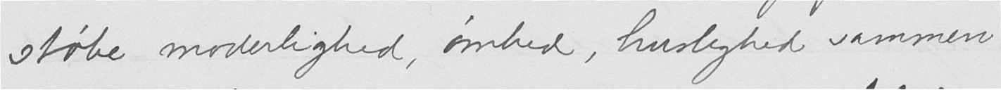støbe moderlighed, ømhed, huslighed sammen
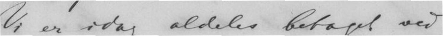Vi er idag aldeles betaget ved
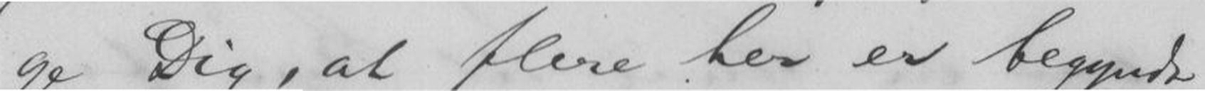ge Dig, at flere her er begyndt
